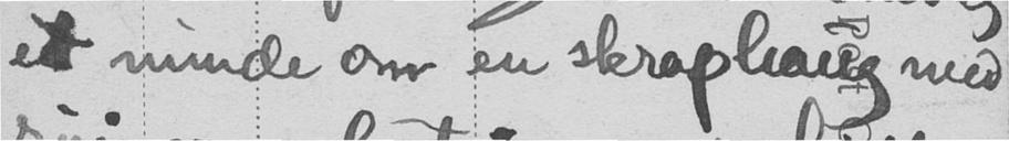et minde om en skraphaug med
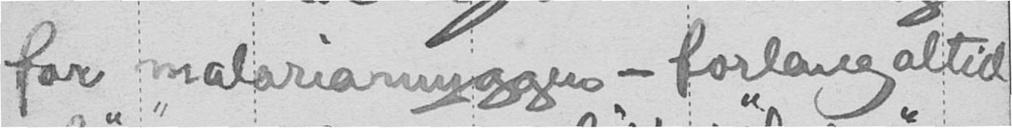for malariamyggen - forlang altid
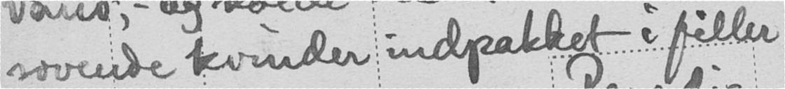sovende kvinder indpakket i filler
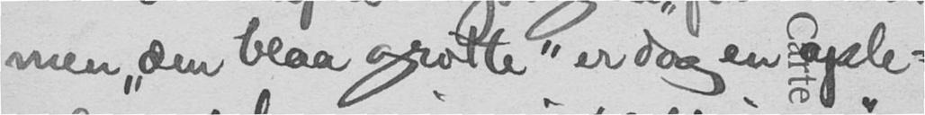men, "den blaa grotte" er dog en ople-
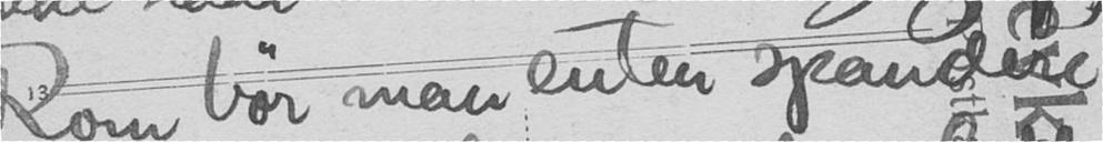Rom bør man enten spandere
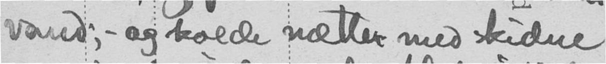vand, - og kolde nætter med skidne
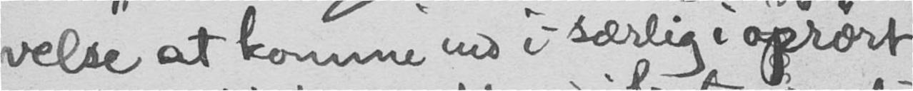velse at komme ind i særlig i oprørt
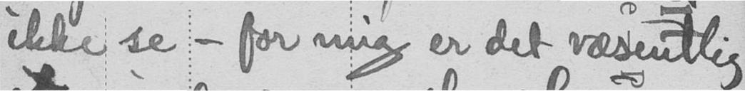ikke se, - for mig er det væsentlig
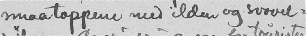smaatoppene med ilden og svovel-
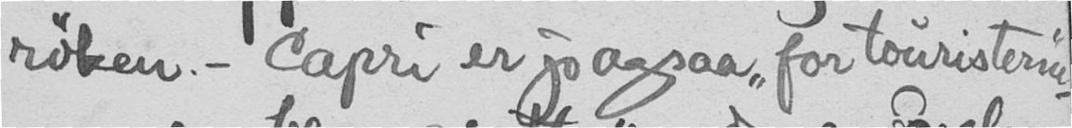røken. - Capri er jo ogsaa "for touristerne",
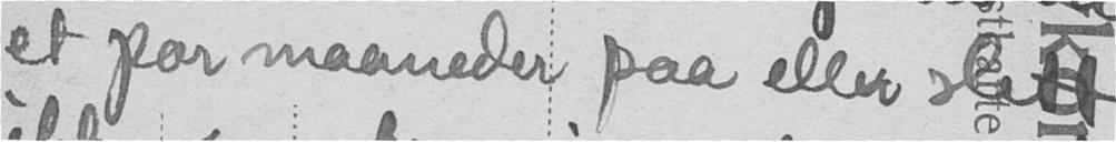et par maaneder paa eller slett
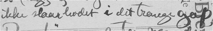ikke slaar hodet i det trange gap).
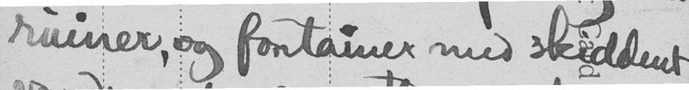ruiner, og fortainer med skiddent
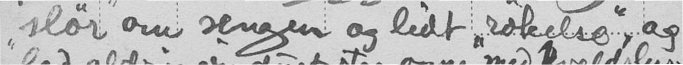"slør" om sengen og lidt "røkelse", og
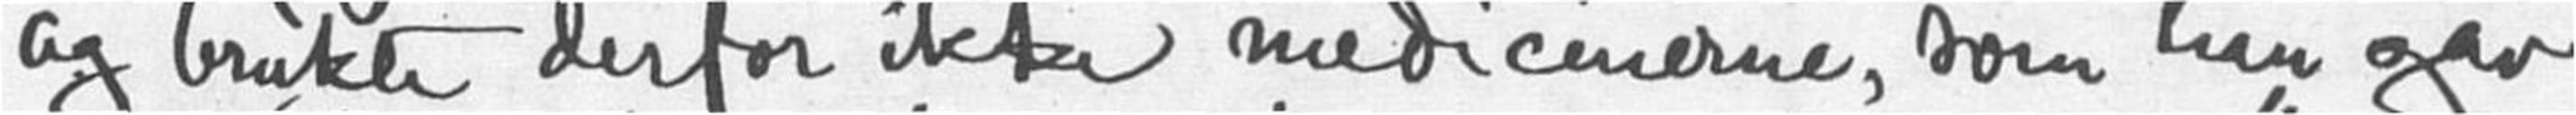og brukte derfor ikke medicinerne, som han gav
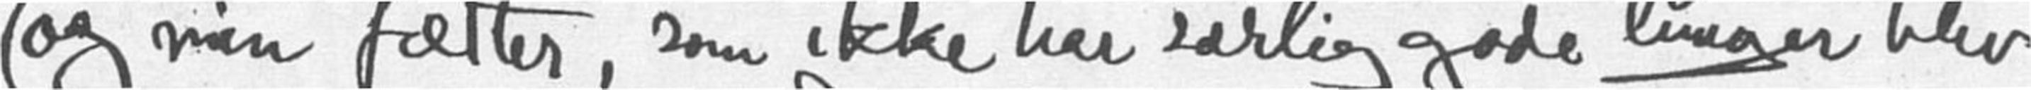(og min fætter, som ikke har særlig gode lunger blev
